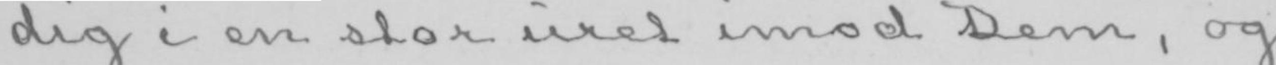dig i en stor uret imod Dem, og
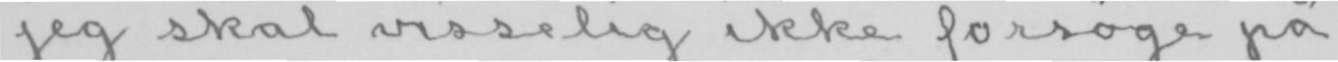jeg skal visselig ikke forsøge på
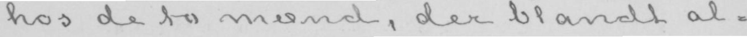hos de to mænd, der blandt al-
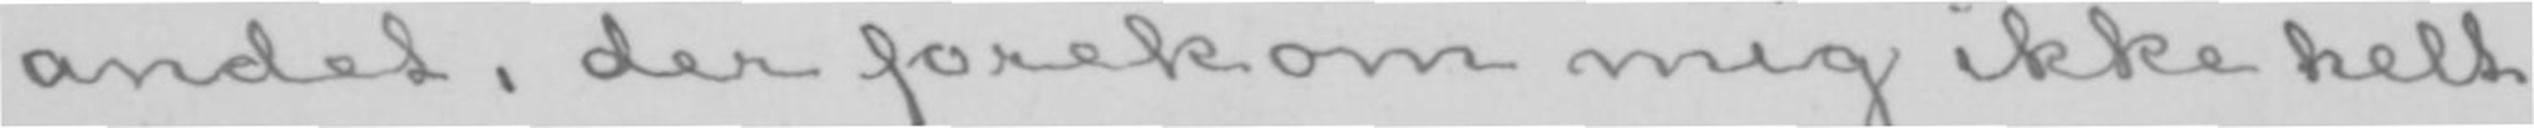andet, der forekom mig ikke helt
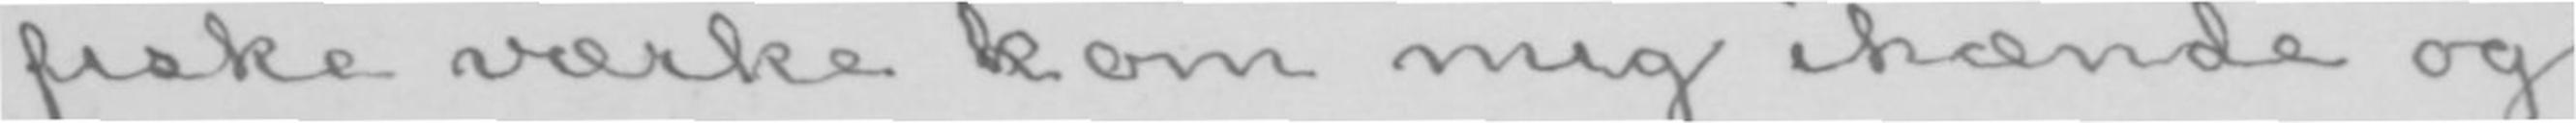fiske værke kom mig ihænde og
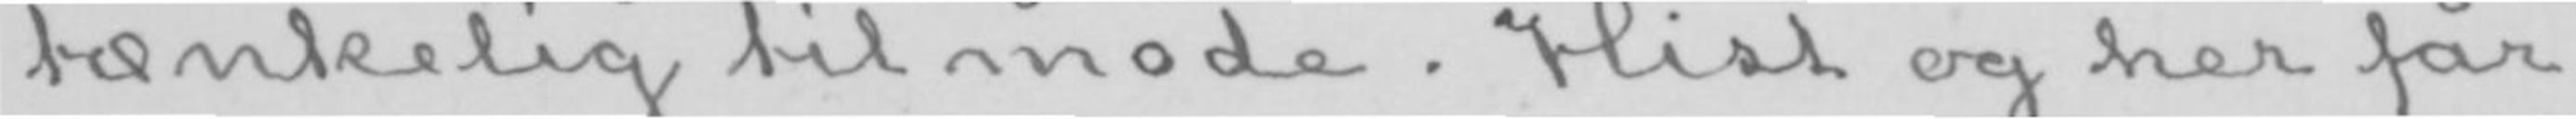tænkelig tilmode. Hist og her får
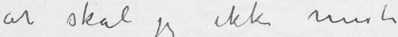at skal jeg ikke miste
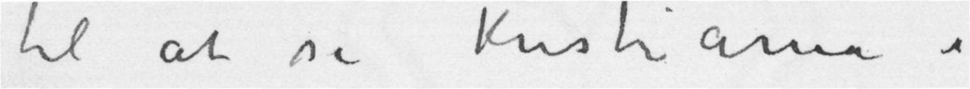til at se Kristiania i
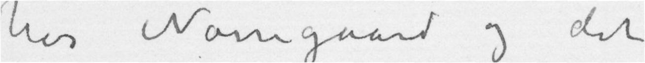hos Nørregaard og det
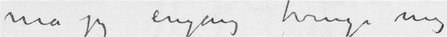må jeg engang tvinge mig
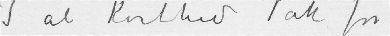I al korthed Tak for
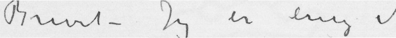Brevet – Jeg er enig i
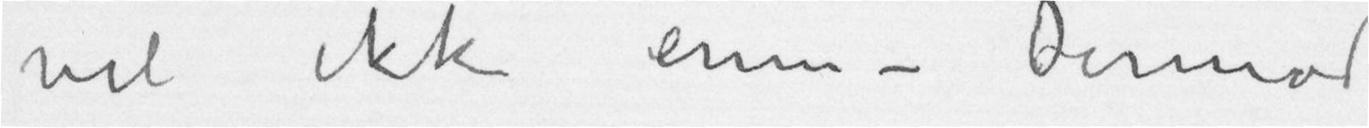vel ikke ennu – Derimod
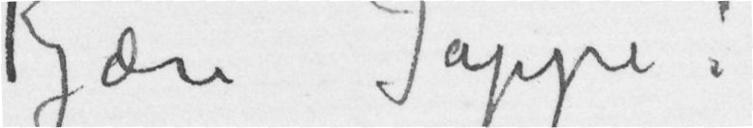Kjære Jappe!
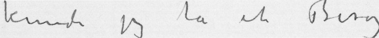kunde jeg ta et Besøg
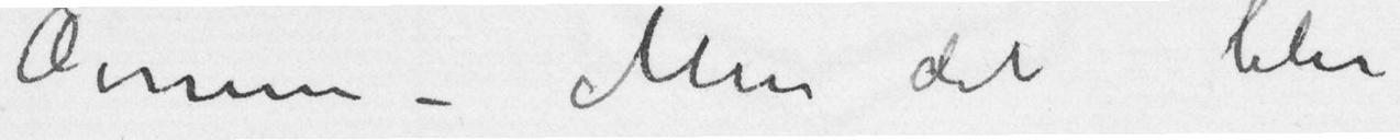Øinene – Men det blir
